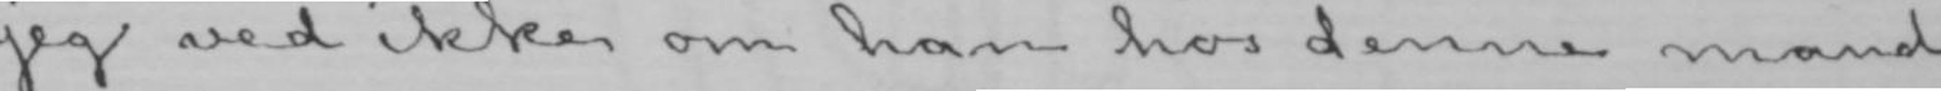jeg ved ikke om han hos denne mand
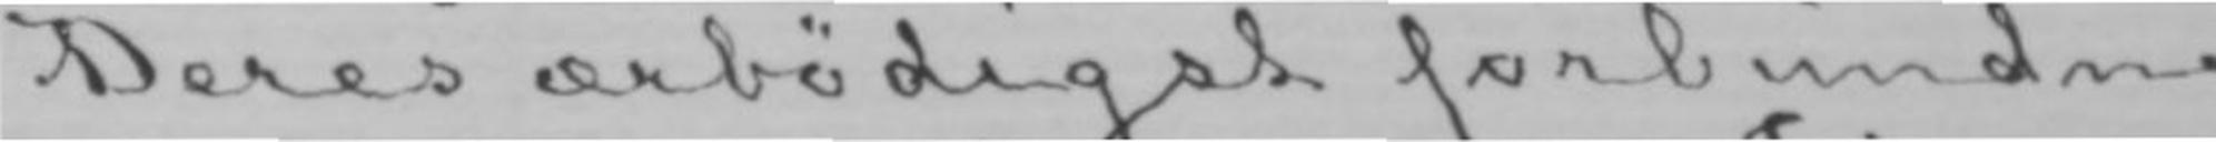Deres ærbødigst forbundne
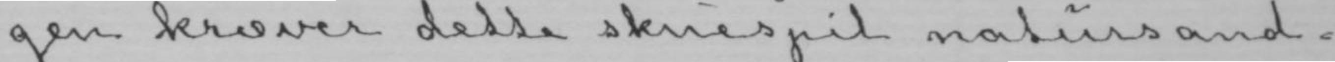gen kræver dette skuespil natursand-
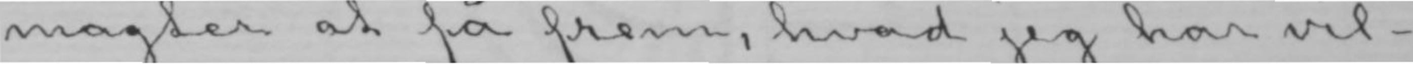magter at få frem, hvad jeg har vil-
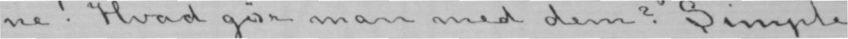ne! Hvad gør man med dem? Simple
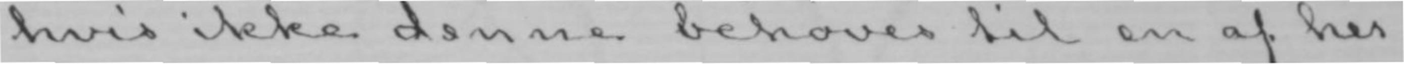hvis ikke denne behøves til en af her-
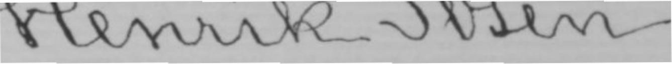Henrik Ibsen.
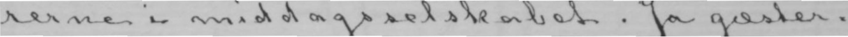rerne i middagsselskabet. Ja gæster-
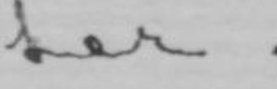ter.
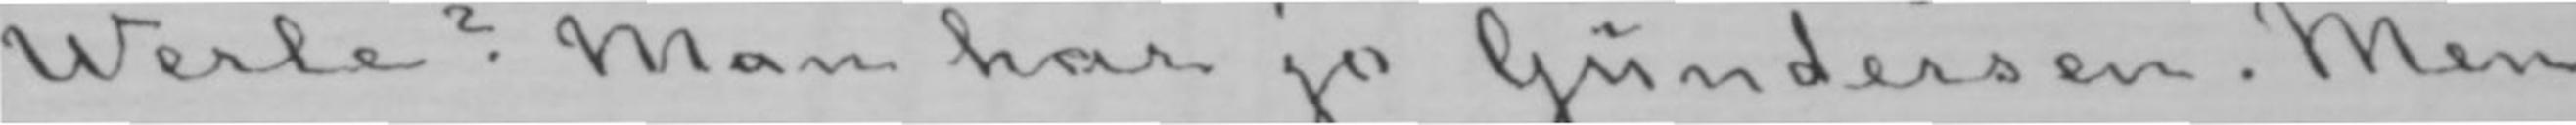Werle? Man har jo Gundersen. Men
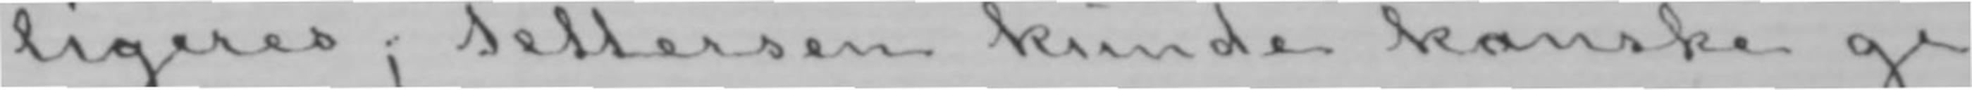ligeres; Pettersen kunde kanske gi-
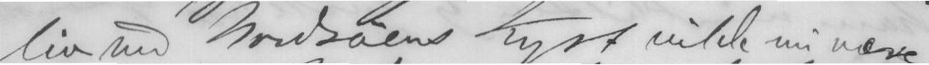liv ved Nordsøens Kyst vilde nu være
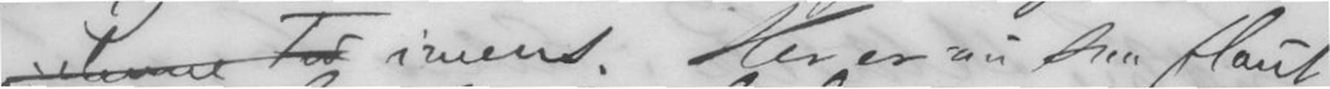i denne Tid imens. Her er nu saa flaut
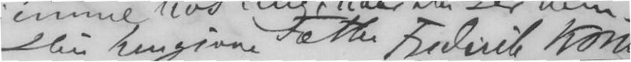Din hengivne Fætter Frederik Konow
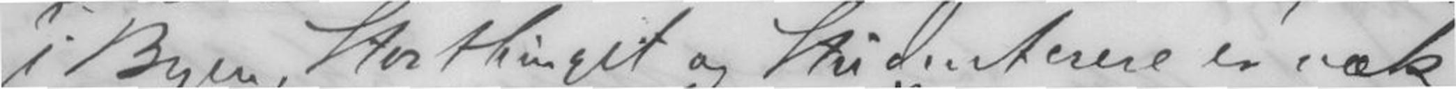i Byen, Storthinget og Studenterne er væk
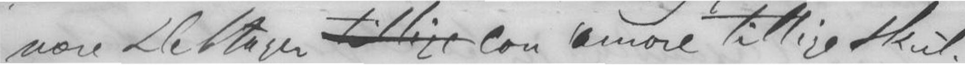være Deltagertillige con amore tillige skul-
